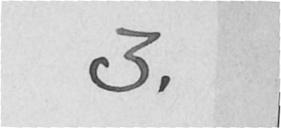3.
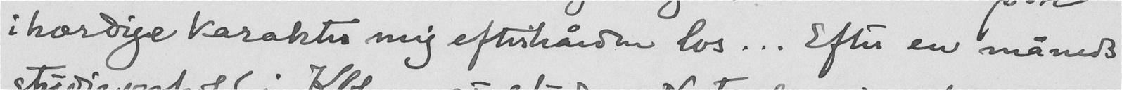ihærdige karakter mig efterhånden los.. Efter en måneds
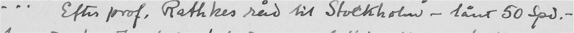... Efter prof, Rathkes råd til Stockholm - lånt 50 spd.-
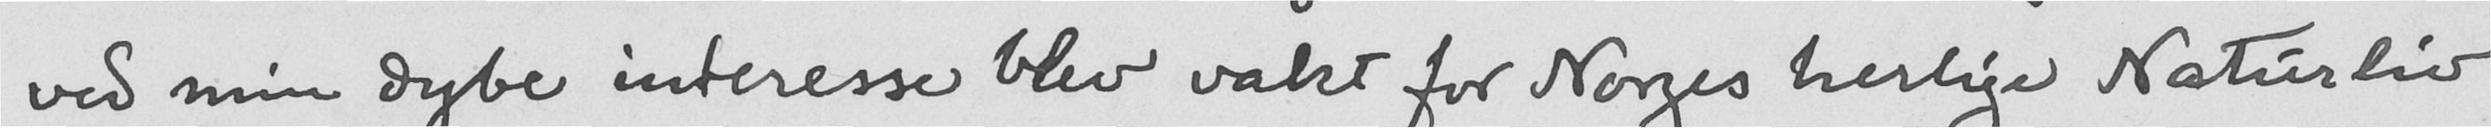ved min dybe interesse blev vakt for Norges herlige Naturliv
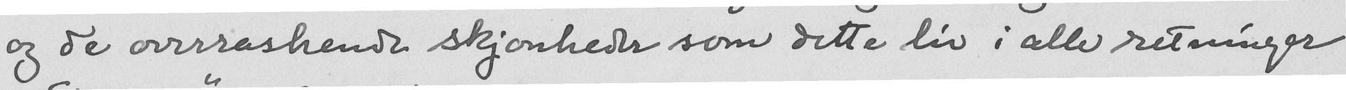og de overraskende skjønheder som dette liv i alle retninger
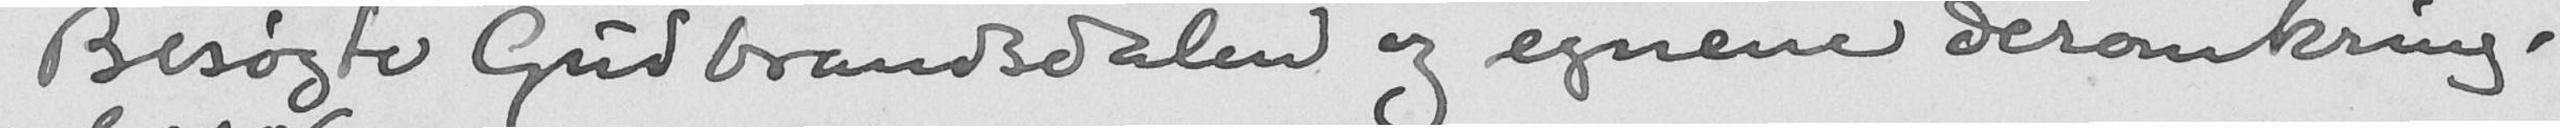Besøgte Gudbrandsdalen og egnene deromkring.
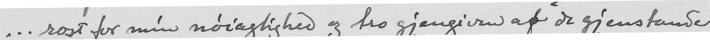...rost for min nøiagtighed og tro gjengiven af de gjenstande
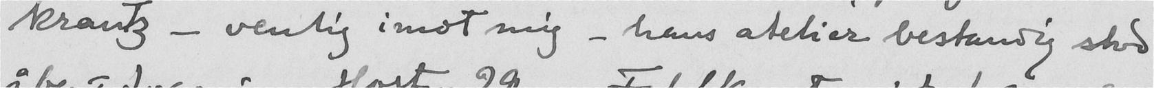krantz - venlig imot mig - hans atelier bestandig stod
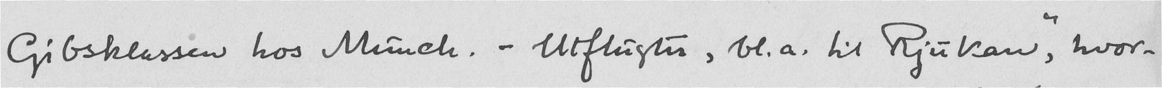Gibsklassen hos Munch. - Utflugter, bl.a. til Rjukan, "hvor-
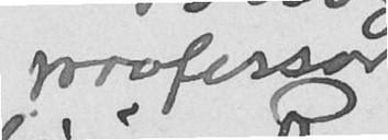professor
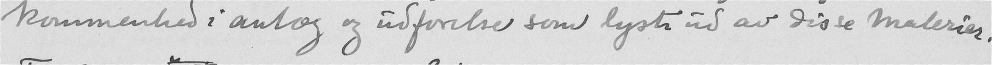kommenhed i anlæg og udførelse som lyste ud av disse malerier.
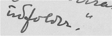udfolder."
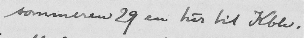sommeren 29 en tur til Kbh.
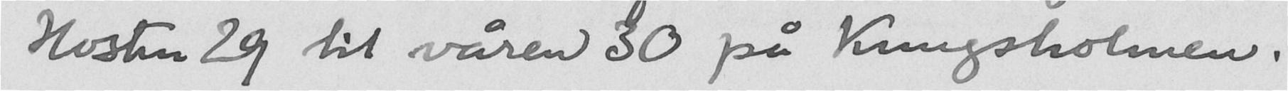Hosten 29 til våren 30 på Kungsholmen.
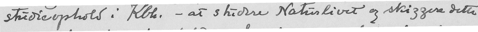studieophold i Kbh. - at studere Naturlivet og skizzere dette
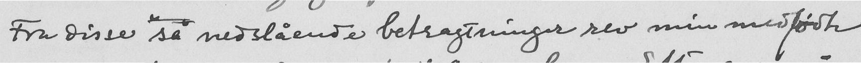Fra disse så nedslående betragtninger rev min medfødte
